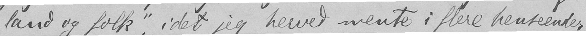land og folk", idet jeg herved mente i flere henseender
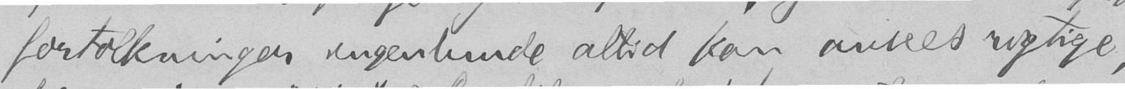fortolkninger ingenlunde altid kan ansees rigtige,
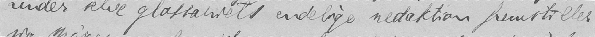under selve glossariets endelige redaktion fremstiller
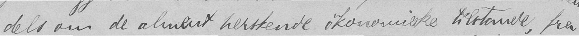dels om de alment herskende økonomiske tilstande, fra
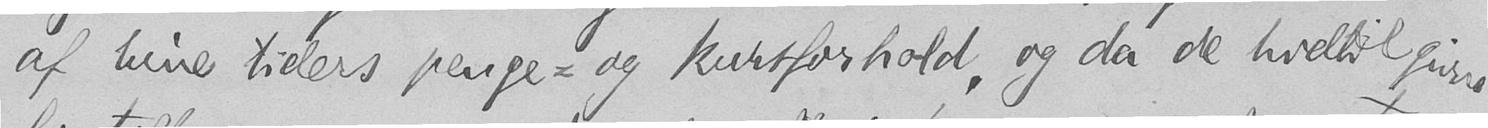af hine tiders penge- og kursforhold, og da de hidtil givne
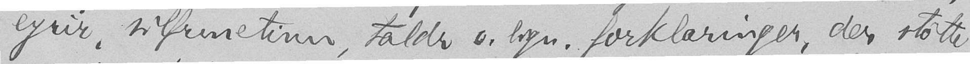eyrir, silfrinetinn, taldr o. lign. forklaringer, der støtte
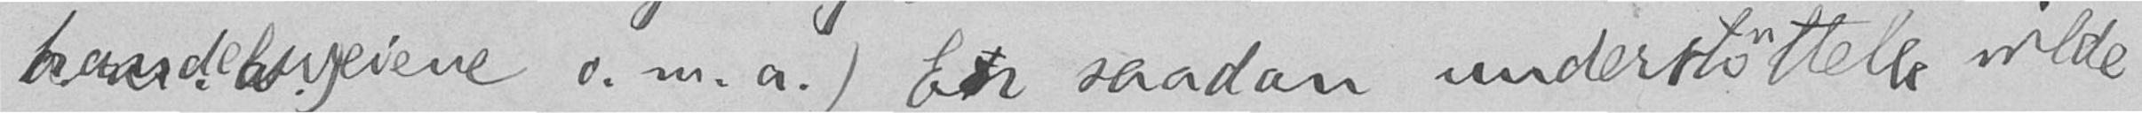handelsveiene o. m. a.) En saadan understøttelse vilde
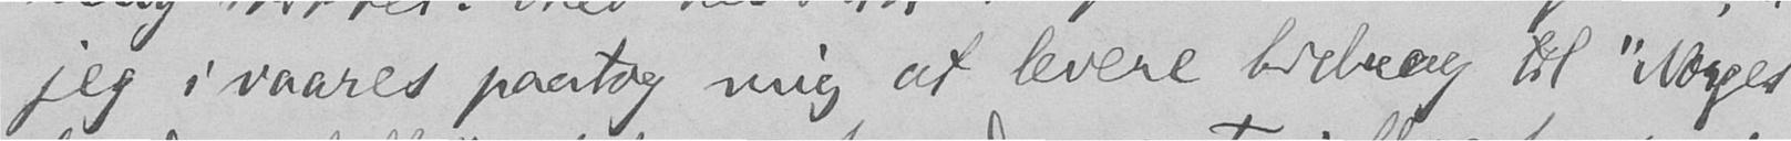jeg i vaares paatog mig at levere bidrag til "Norges
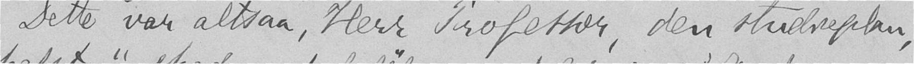Dette var altsaa, Herr Professor, den studieplan,
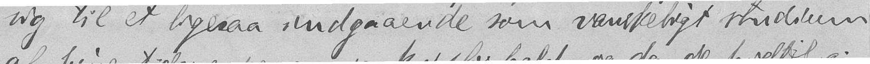sig til et ligesaa indgaaende som vanskeligt studium
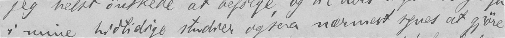i mine hidtidige studier ogsaa nærmest synes at gjøre
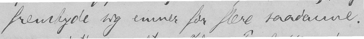frembyde sig emner for flere saadanne.
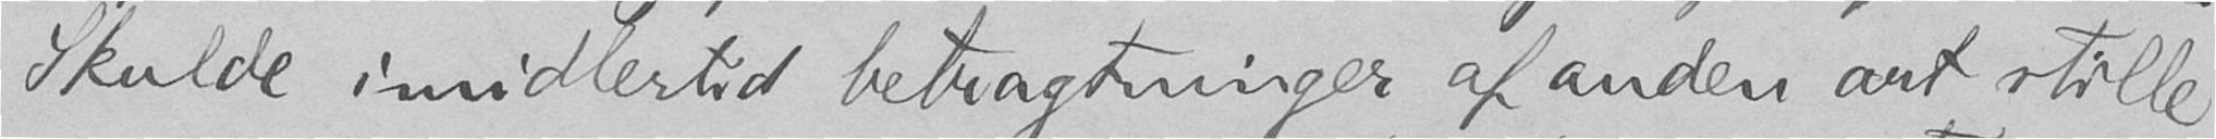Skulde imidlertid betragtninger af anden art stille
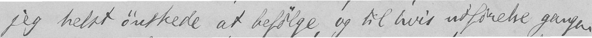jeg helst ønskede at befølge, og til hvis udførelse gangen
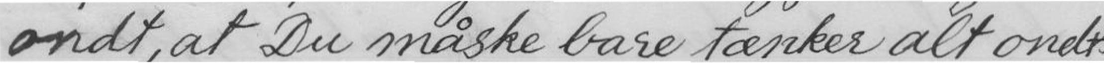ondt, at Du måske bare tænker alt ondt
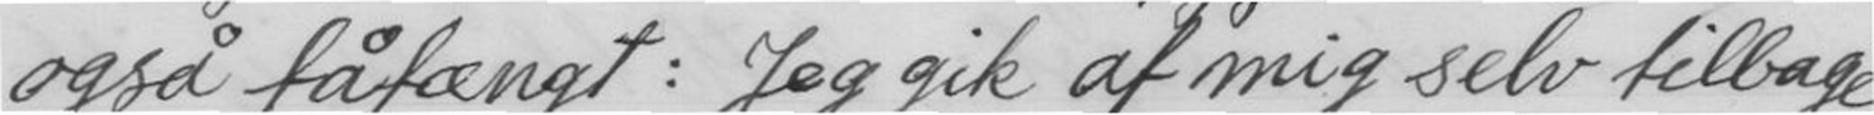også fåfængt: Jeg gik af mig selv tilbage
